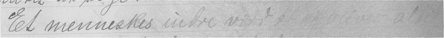Et menneskes indre værd er og bliver altid
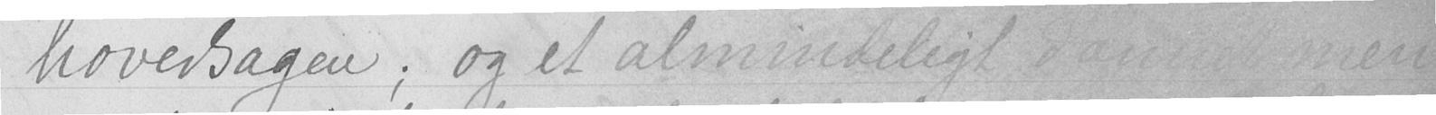hovedsagen; og et almindeligt dannet men-
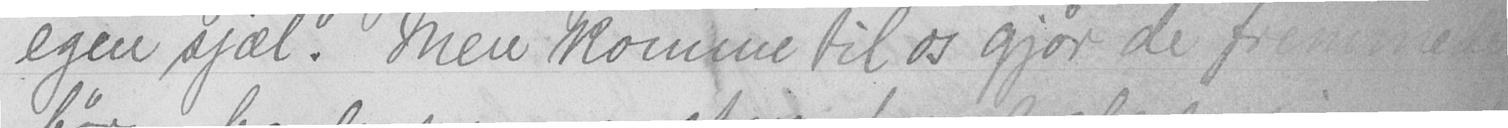egen sjæl". Men komme til os gjør de fremmede
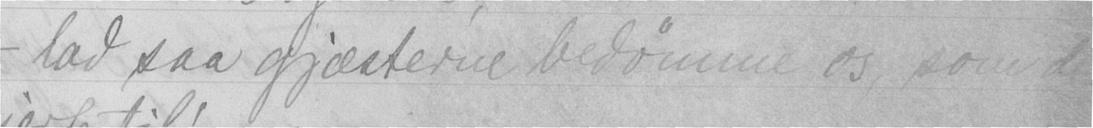- lad saa gjæsterne bedømme os, som de
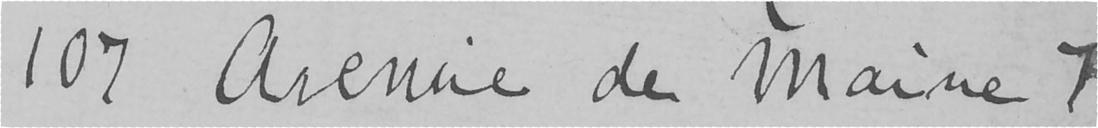107 Avenue de Maine 7
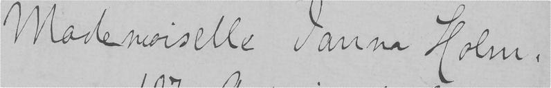Mademoiselle Janna Holm.
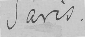Paris.
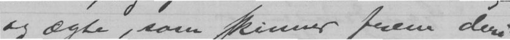og ægte, som skinner frem deri-
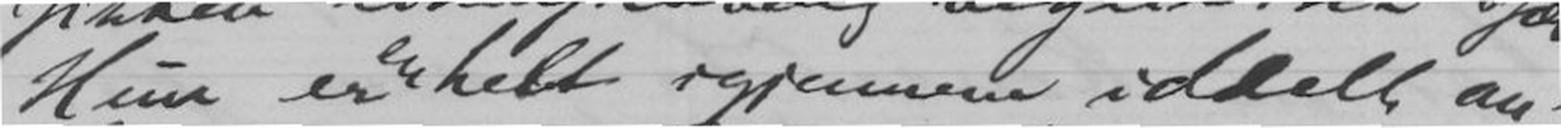Hun er en helt igjennem ideelt an-
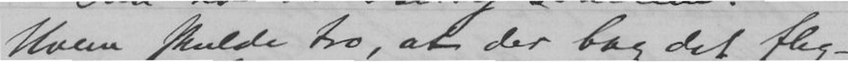Hvem skulde tro, at der bag det fleg-
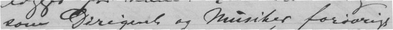som Dirigent og Musiker forøvrigt
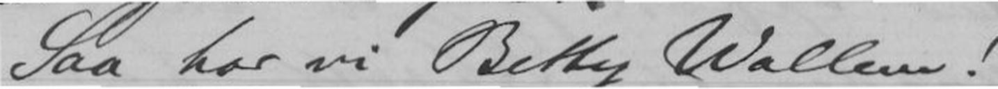Saa har vi Betty Wallem!
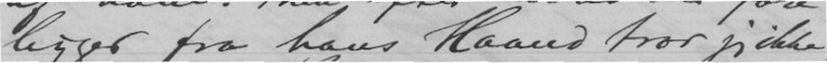ligger fra hans Haand tror jeg ikke,
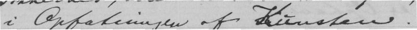i Opfatningen af Kunsten. -
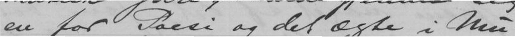en for Poesi og det ægte i Mu-
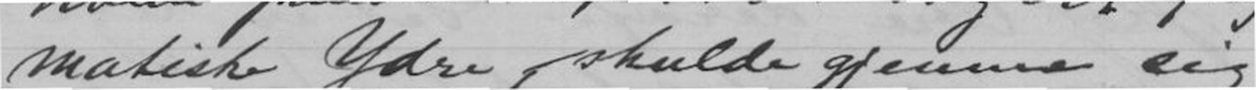matiske Ydre, skulde gjemme sig
